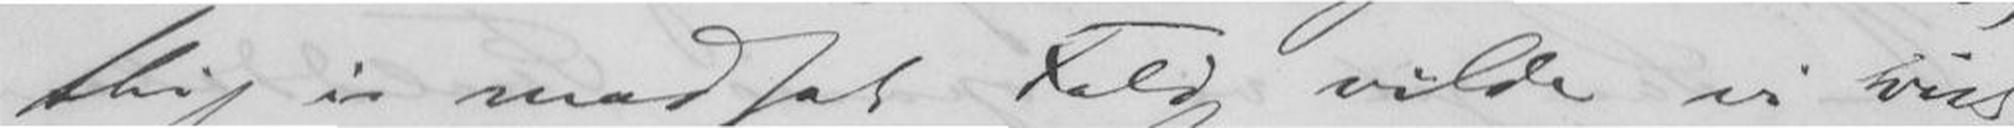thi i modsat Fald vilde vi vist
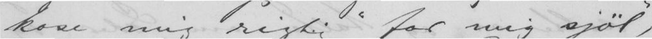kose mig rigtig "for mig sjøl",
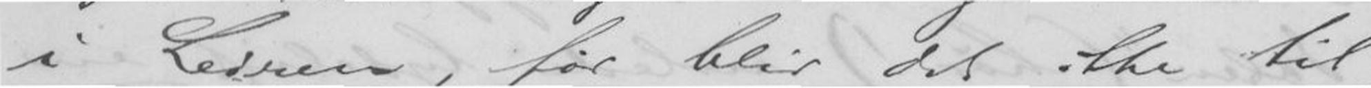i Leiren, før blir det ikke til
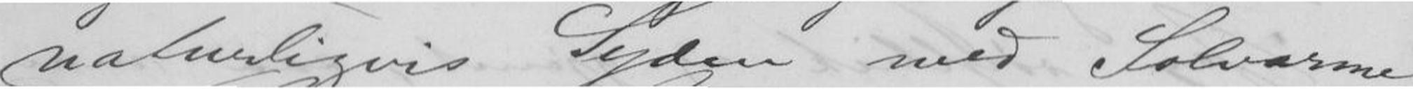naturligvis Syden med Solvarme
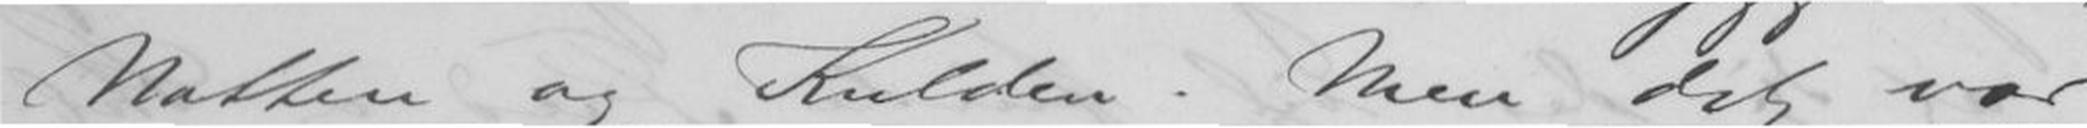Natten og Kulden. Men det var
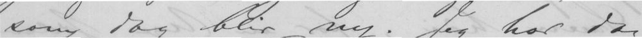som dog blir ny. Jeg har dog
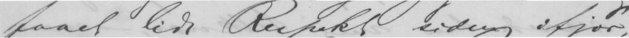faaet lidt Respekt siden ifjor,
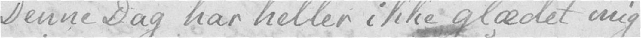Denne Dag har heller ikke glædet mig
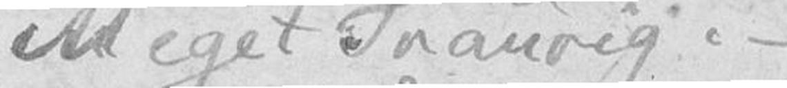Meget Traurig. –
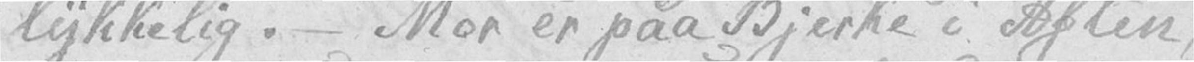lykkelig. – Mor er paa Bjerke i Aften,
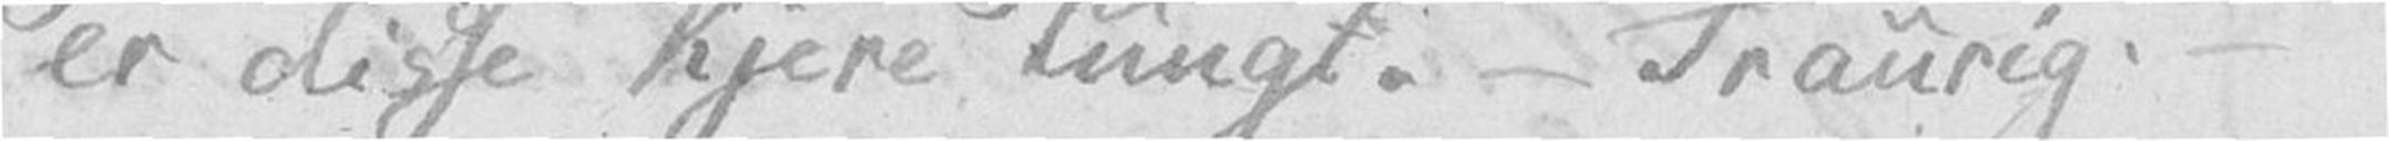er disse kjere tungt. – Traurig. –
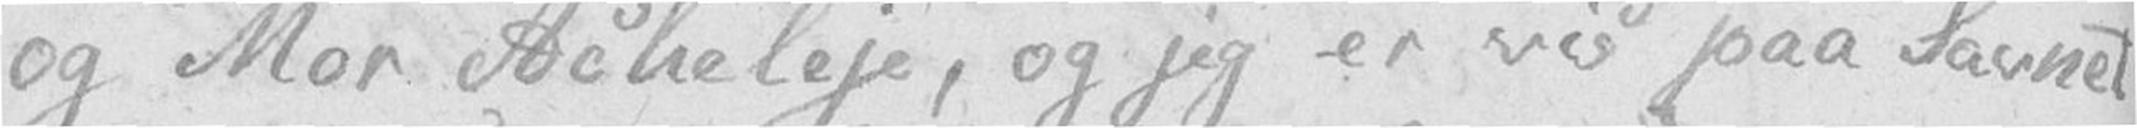og Mor Acheleje, og jeg er vis paa Savnet
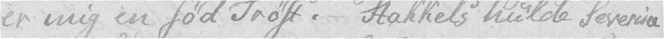er mig en sød Trøst. – Stakkels hulde Severine
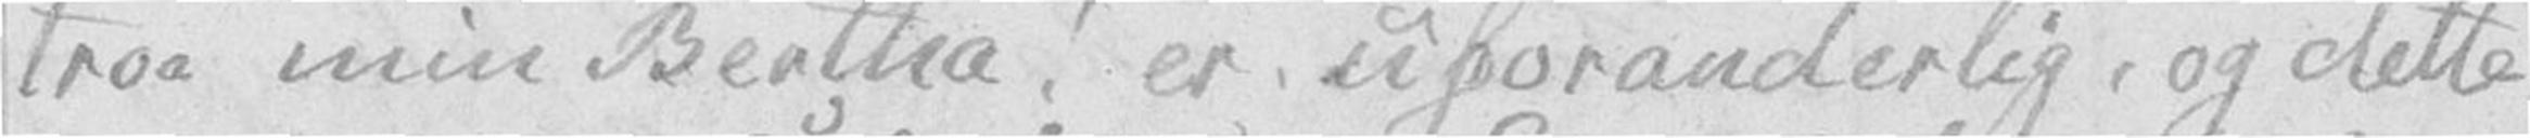troe min Bertha! er uforanderlig, og dette
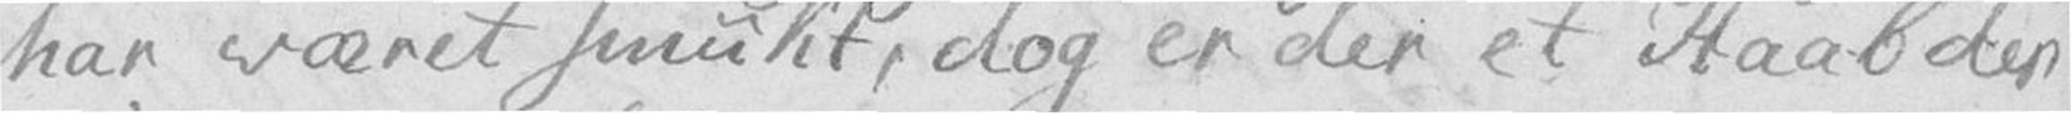har været smukt, dog er der et Haab der
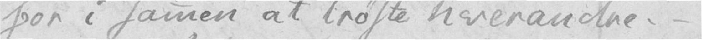for i sammen at trøste hverandre. –
